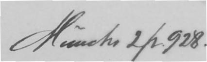Munch 2/2 928.
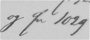og fr 1029
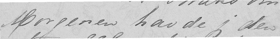Morgenen havde jeg den
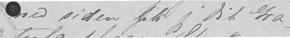red siden fik jeg dit Gra-
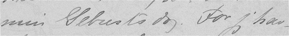min Geburtsdag. For jeg hav-
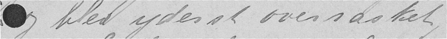og blev yderst overrasket,
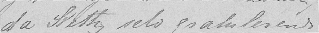da Kitty selv gratulerende
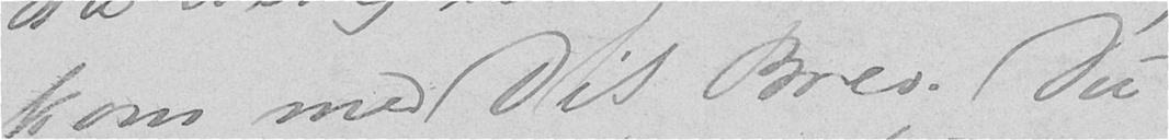kom med Dit Brev. Du
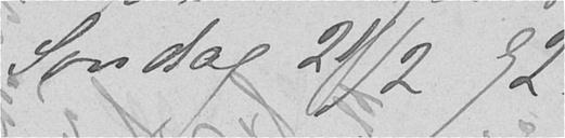Søndag 21/2 92
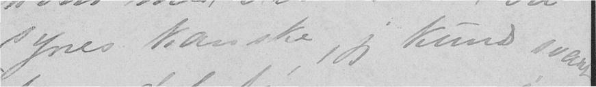synes kanske, jeg kunde svaret
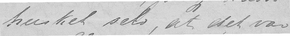husket selv, at det var
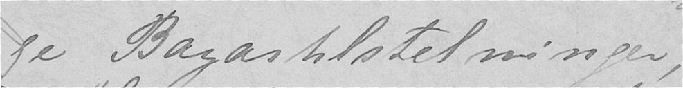ge Bazartilstelninger,
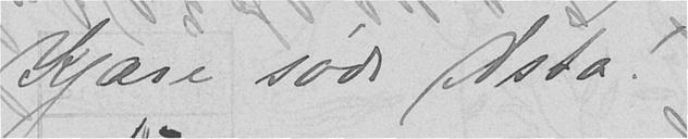Kjære søde Asta.
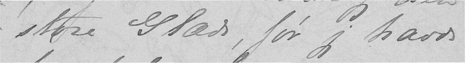store Glæde, før jeg havde
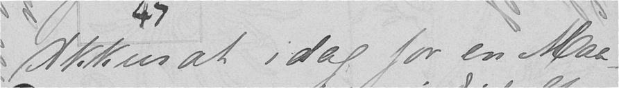Akkurat i dag for en Maa-
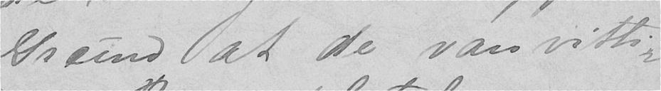Grund at de vanvitti-
